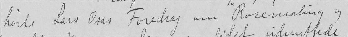hørte Lars Osas Foredrag om "Rosemaling" og
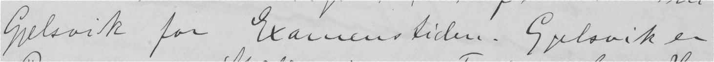Gjelsvik for Examenstiden. Gjelsvik er
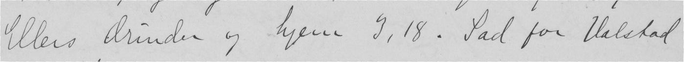Ellers Ærinder og hjem 3, 18. Sad for Valstad
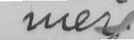mer.
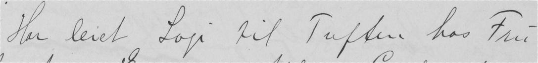Har leiet Logi til Tuften hos Fru
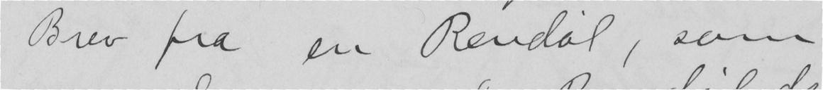Brev fra en Rendal, som
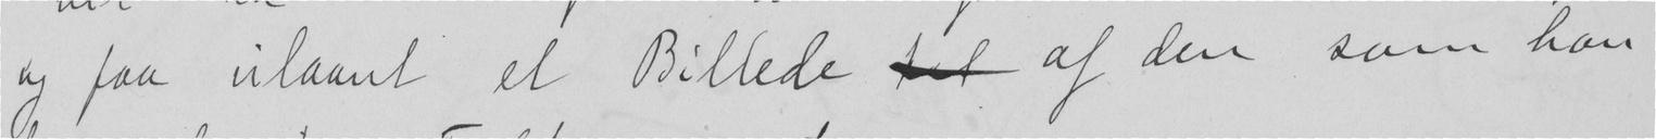og faa ulaant et Billede  af den som han
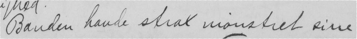Banden havde strax mønstret sine
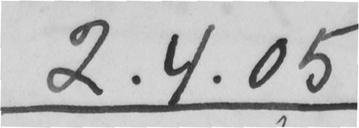2.4.05
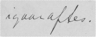igaaraftes.
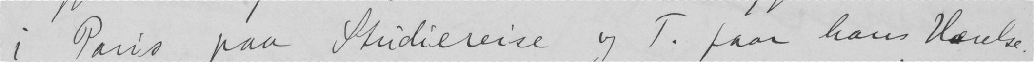i Paris paa Studiereise og T. faar hans Værelse.
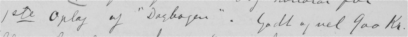1ste Oplag af "Dagbogen". Godt og vel 900 Kr.
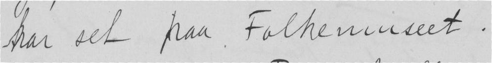har set paa Folkemuseet.
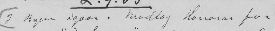I Byen igaar. Modtog Honorar for
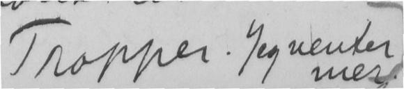Tropper. Jeg venter
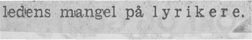ledens mangel på lyrikere.
